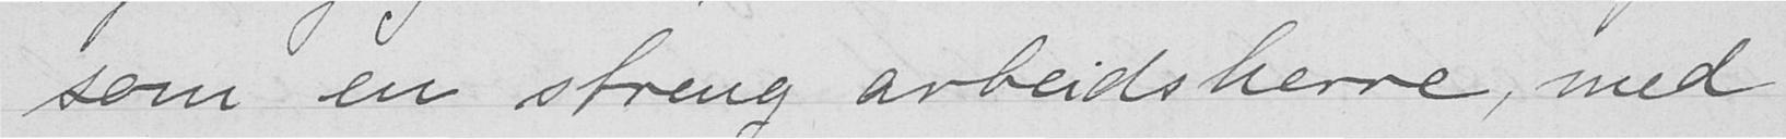som en streng arbeidsherre, med
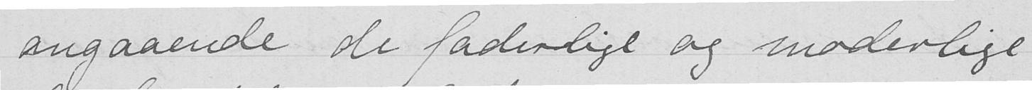angaaende de faderlige og moderlige
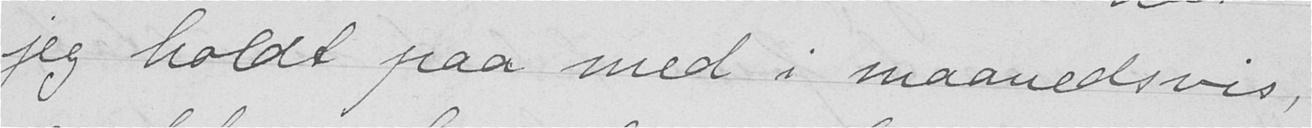jeg holdt paa med i maanedsvis,
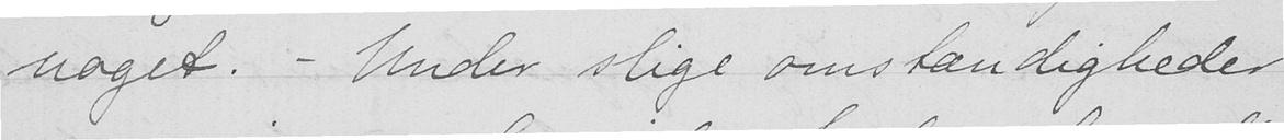noget. - Under slige omstændigheder
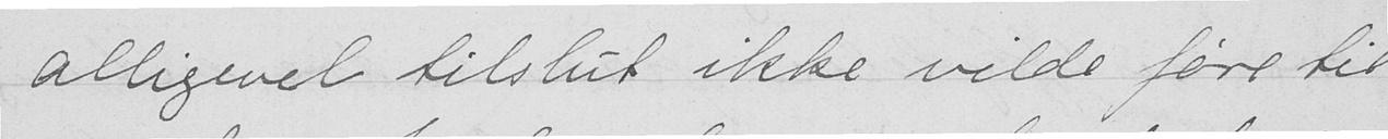alligevel tilslut ikke vilde føre til
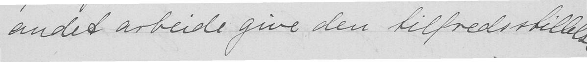andet arbeide give den tilfredsstillelse,
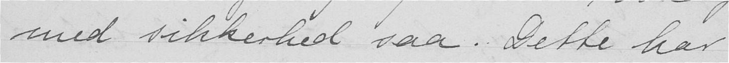med sikkerhed saa. Dette har
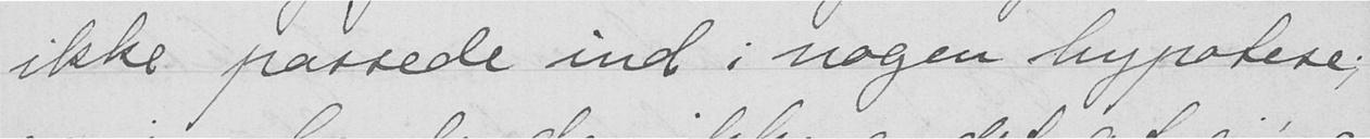ikke passede ind i nogen hypotese;
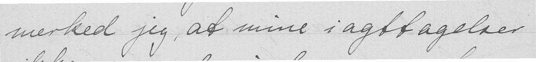merked jeg, at mine iagttagelser
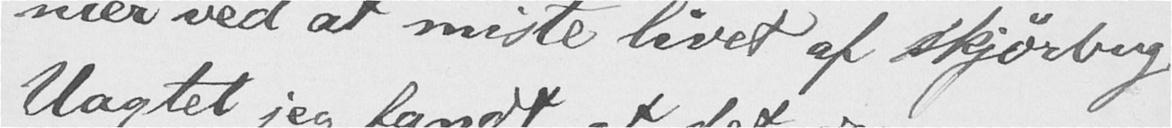nær ved at miste livet af skjørbug.
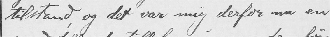tilstand, og det var mig derfor nu en
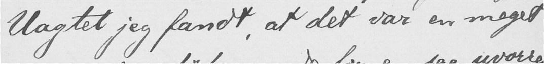Uagtet jeg fandt, at det var en meget
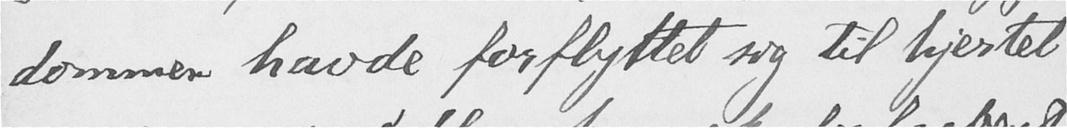dommen havde forflyttet sig til hjertet
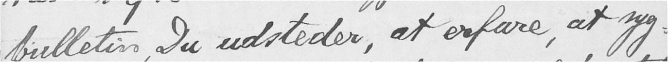bulletin, Du udsteder, at erfare, at syg-
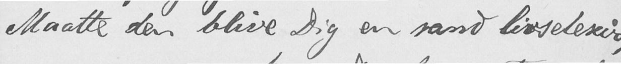Maatte den blive Dig en sand livselexir,
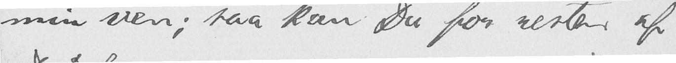min ven; saa kan Du for resten af
